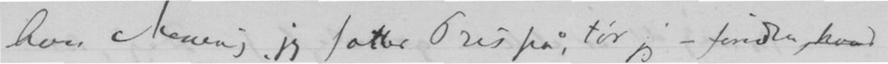hva, Mening jeg sætter Pris på, tør jeg - finder hvad
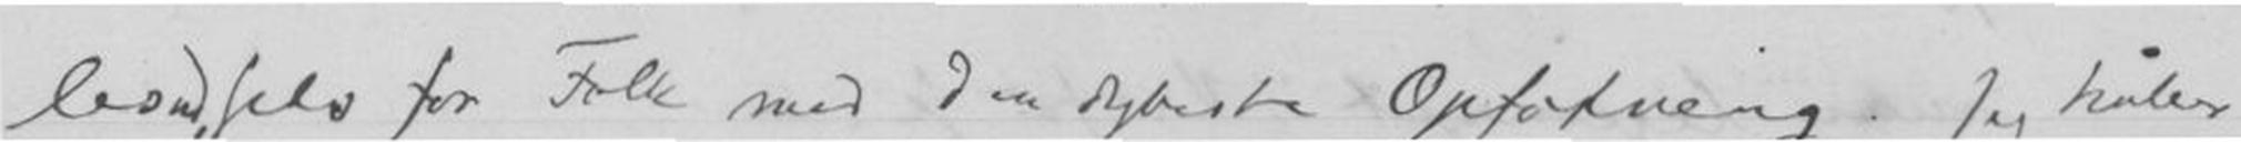les ud, selv for Folk med den dybeste Opfatning. Jeg håber
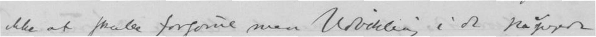ikke at skulle forsøme min Udvikling i de Negative
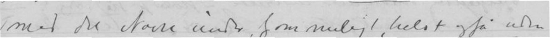(med det Navn under, som muligt, helst også uden
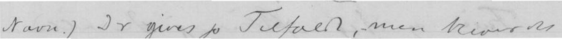Navn). Der gives jo Tilfælde, men skriver
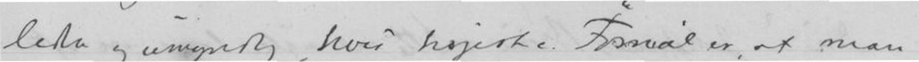liten og umyndig, hvis højeste Formål er at man
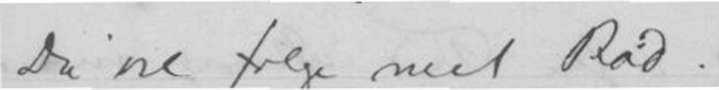Du vil følge mit Råd.
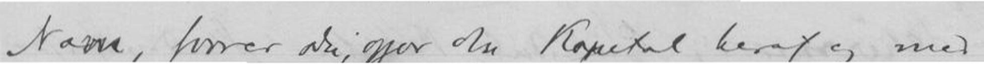Navn, svarer Du, gjør du Kapital heraf og med
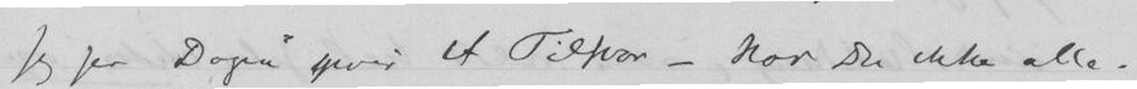Jeg ser "Dagen" spires et Tilsvar - har Du ikke alle-
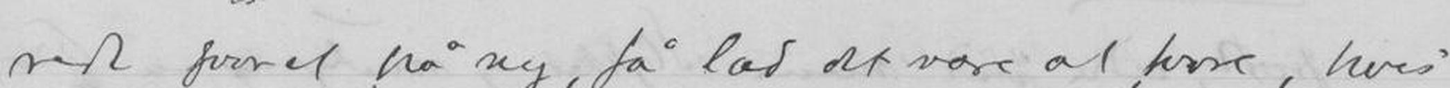rede svaret på ny, så lad det være at svare, hvis
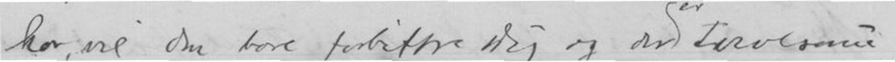har, vil du bare forbittre Dig og der er tvivlsom
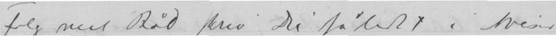følg mit Råd skriv Du så lidet i Avisen
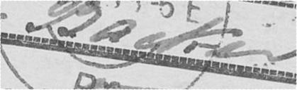Backer
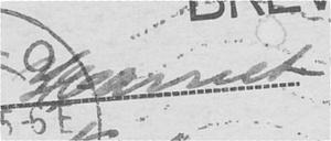Harriet
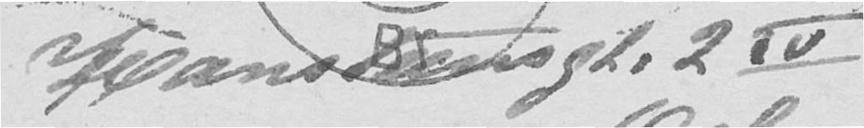Hansteensgt. 2 IV
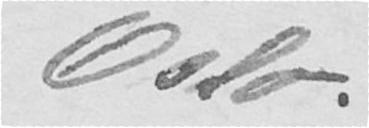Oslo.
